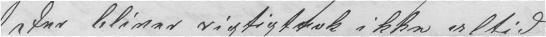Denbliver rigtignok ikke altid
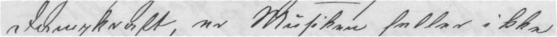Dampkraft, er Musiken heller ikke
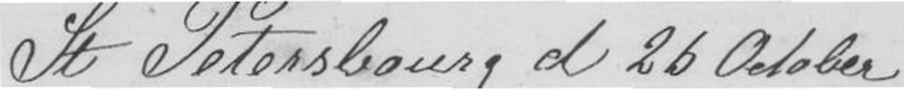St Petersbourg d 26 October
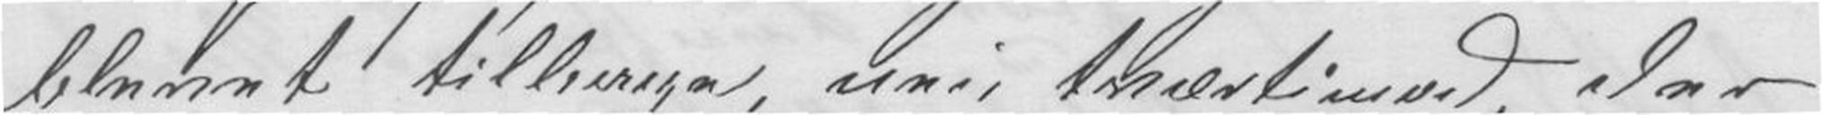blevet tilbage, nei tvætimod, der
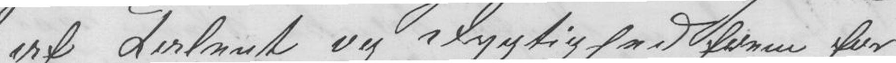af Talent og Dygtighed frem for
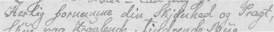Herlig fornemme din Skjønhed og Pragt,
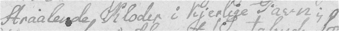Straalende, Kloder i kjerlige Favn
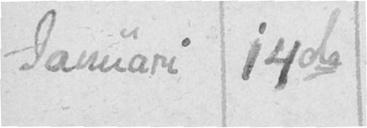Januari 14de
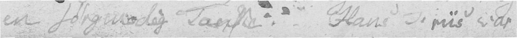en sørgmodig Tanke. Hans Friis var
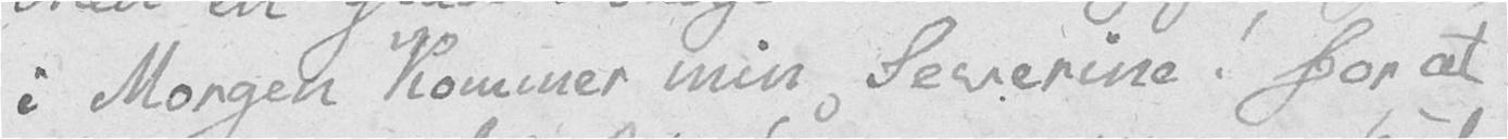i Morgen kommer min Severine! for at
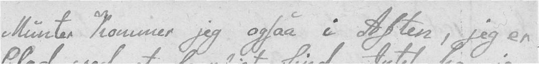Munter kommer jeg ogsaa i Aften, jeg er
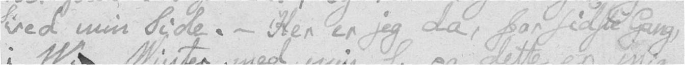ved min Side. – Her er jeg da, for sidste Gang,
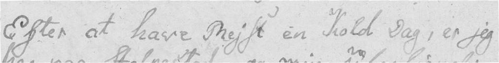Efter at have Rejst en Kold Dag, er jeg
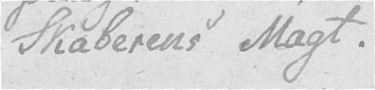Skaberens Magt.
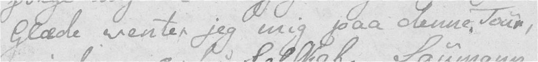Glæde venter jeg mig paa denne Tour,
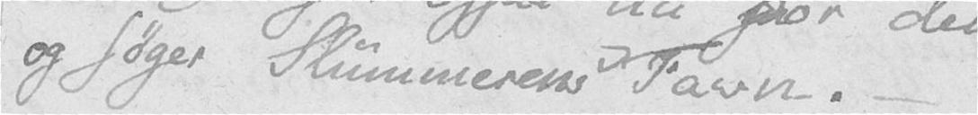og søger Slummerens Favn. –
